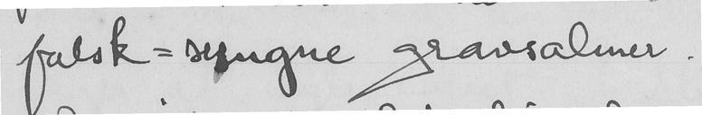falsk-sungne gravsalmer.
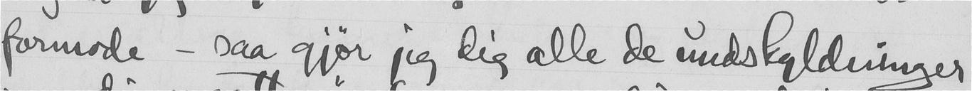formode - saa gjør jeg dig alle de undskyldninger
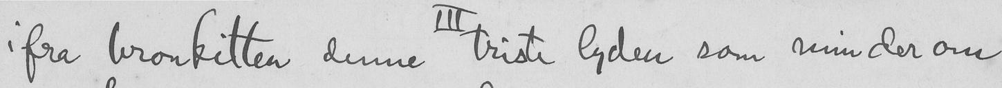ifra bronkitten denne triste lyden som minder om
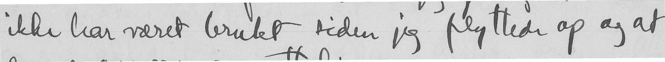ikke har været brukt siden jeg flyttede op og at
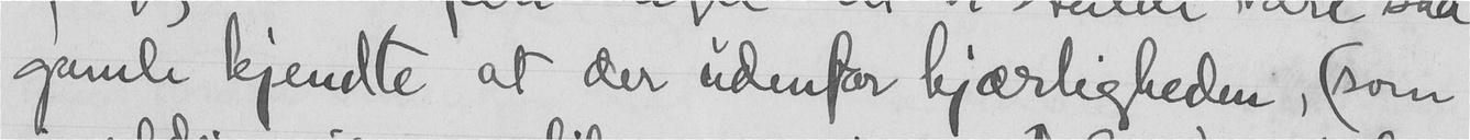gamle kjendte at der udenfor kjærligheden, (som
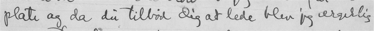plate og da du tilbød dig at lede blev jeg ærgerlig
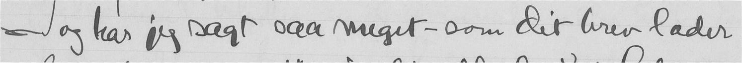og har jeg sagt saa meget - som det brev lader
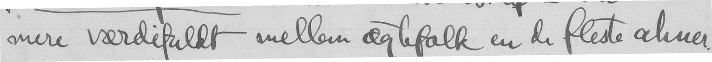mere værdifuldt mellem ægtefolk en de fleste ahner.
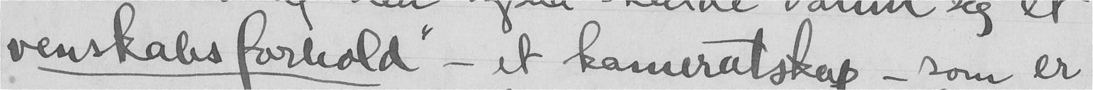venskabsforhold - et kameratskap - som er
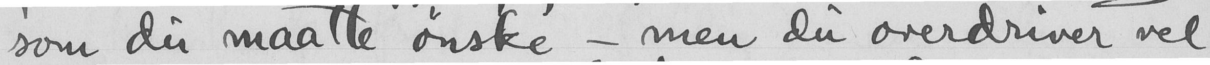som du maatte ønske - men du overdriver vel
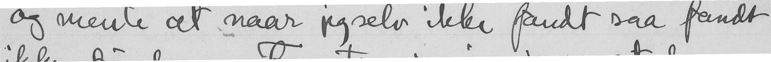og mente at naar jeg selv ikke fandt saa fandt
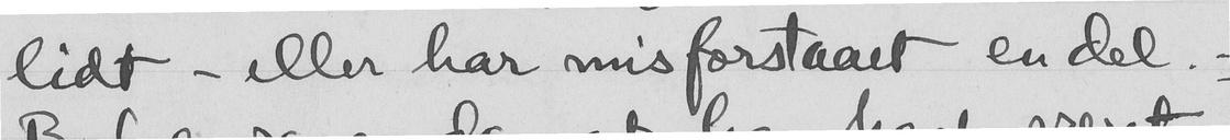lidt - eller har misforstaaet en del. -
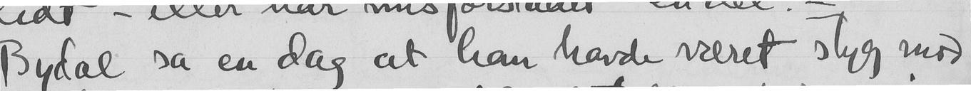Bydal sa en dag at han havde været styg mod
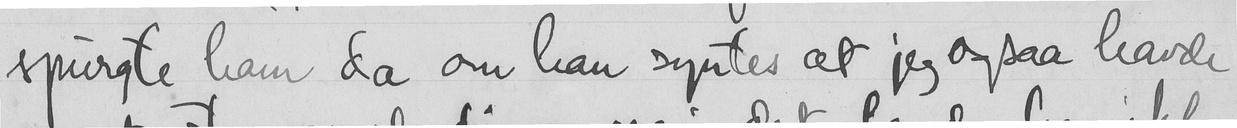spurgte ham da om han syntes at jeg ogsaa havde
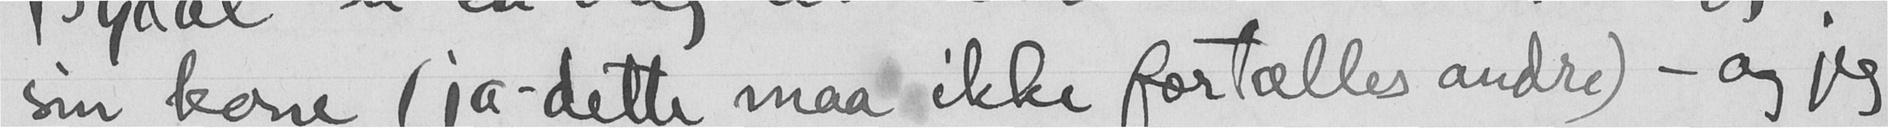sin kone (jo dette maa ikke fortælles andre) - og jeg
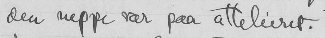den neppe var paa attelieret.
